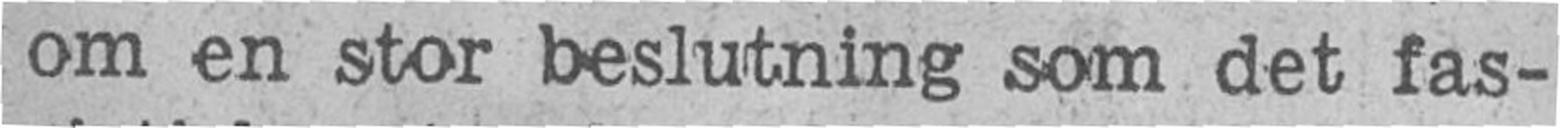om en stor beslutning som det fas-
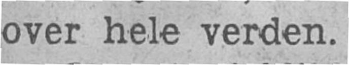over hele verden.
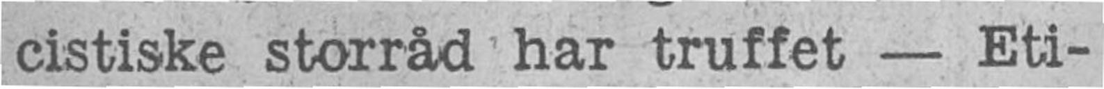cistiske storråd har truffet - Eti-
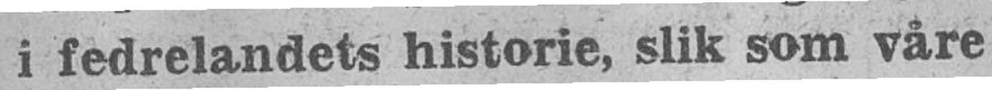i fedrelandets historie, slik som våre
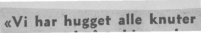"Vi har hugget alle knuter
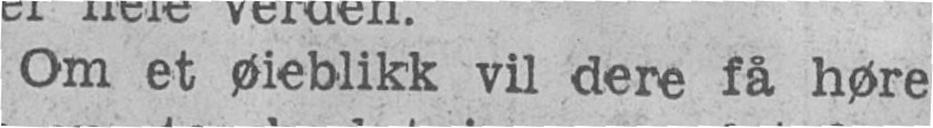Om et øieblikk vil dere få høre
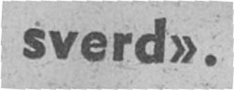sverd".
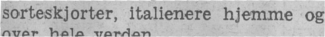sorteskjorter, italienere hjemme og
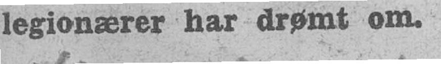legionærer har drømt om.
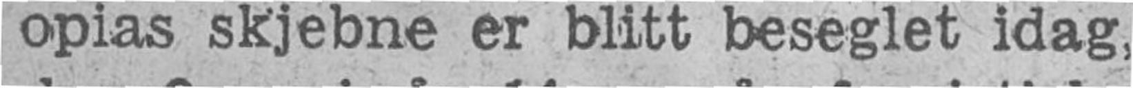opias skjebne er blitt beseglet idag,
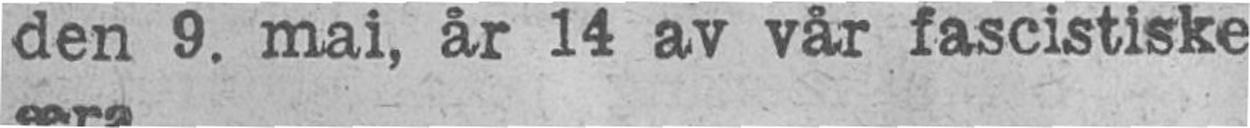den 9. mai, år 14 av vår fascistiske
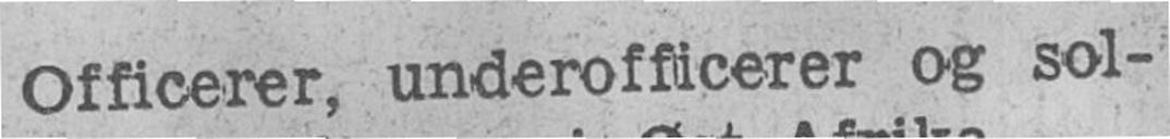Officerer, underofficerer og sol-
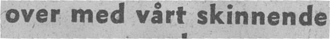over med vårt skinnende
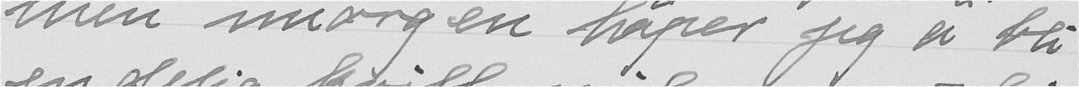men imorgen håper jeg å bli
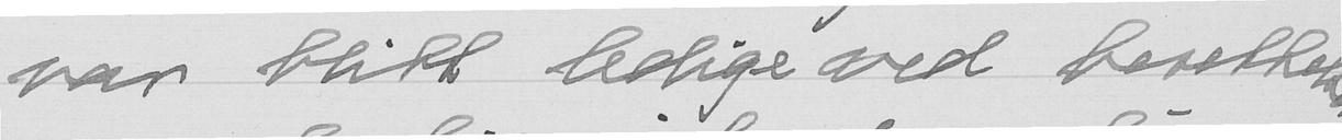var blitt ledige ved besettelsen
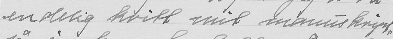endelig kvitt mit manuskript,
